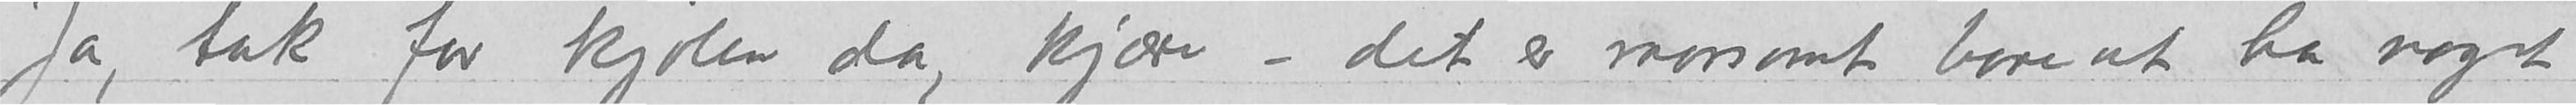Ja, tak for kjolen da, kjære – det er morsomt bare at ha noget
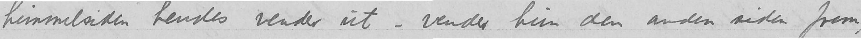himmelsiden hendes vender ut – vender hun den anden siden frem,
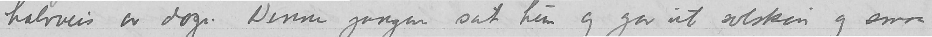halvveis av dage. Denne gangen sat hun og gav ut solskinn og smaa
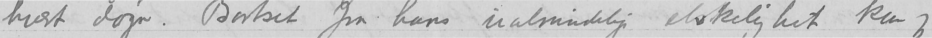hvert døgn.  Bortset fra hans ualmindelige elskelighet kan jeg
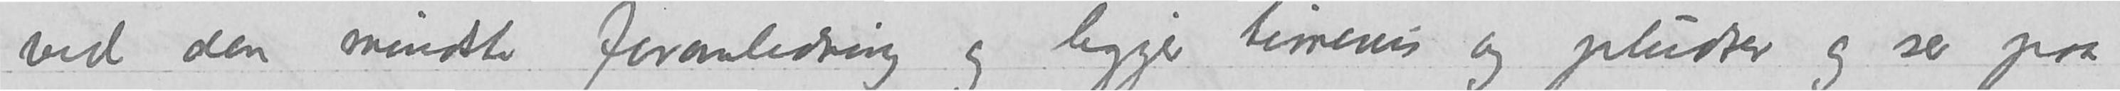ved den mindste foranledning og ligger timevis og pludrer og ser paa
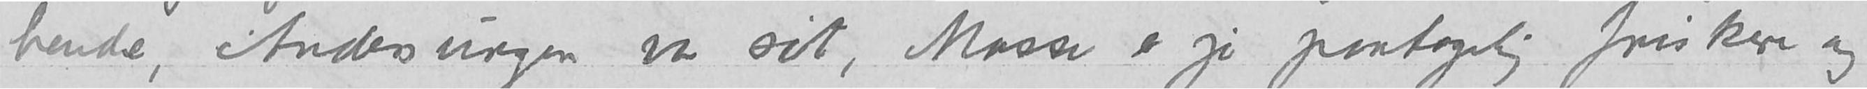hende, Andersungen var søt, Mosse er jo paatagelig friskere og
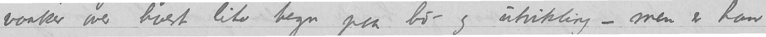vaaker over hvert lite tegn paa liv –- og utvikling – men er han
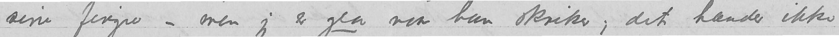sine fingre – men jeg er gla naar han skriker; det hænder ikke
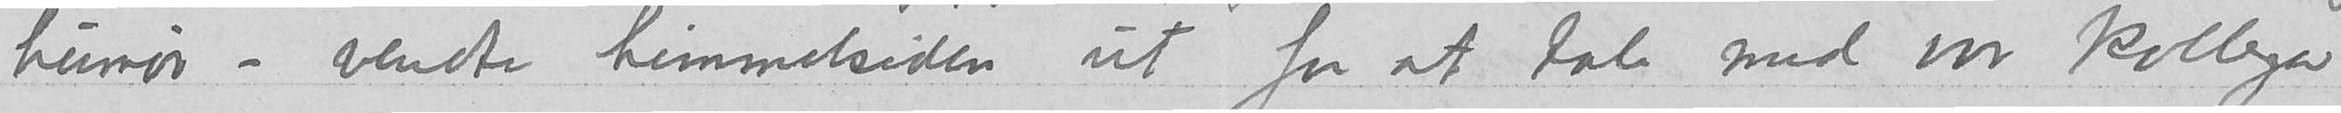humør – vendte himmelsiden ut for at tale med vor kollega
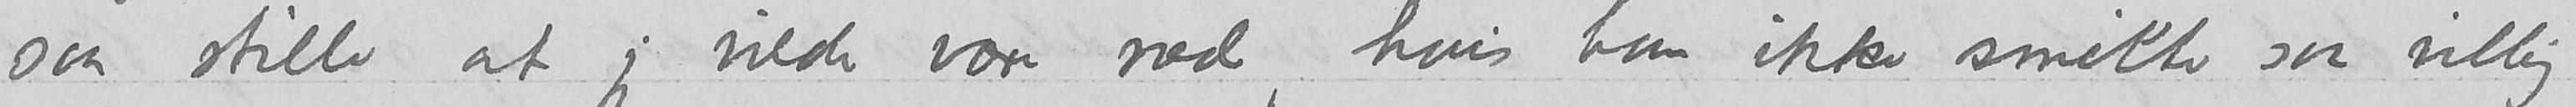saa stille at jeg vilde være ræd, hvis han ikke smilte saa villig
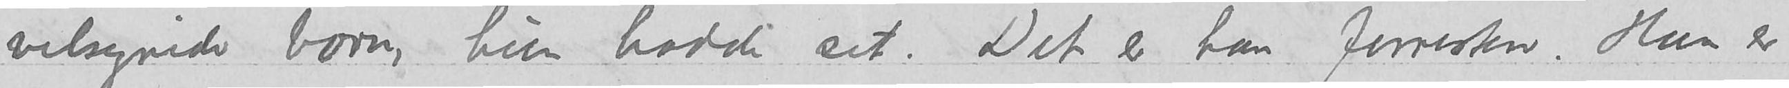velsignede barn, hun hadde set.  Det er han forresten.  Han er
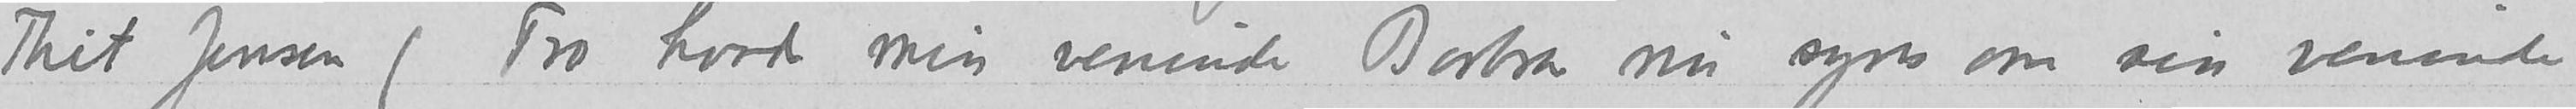Thit Jensen (Tro hvad min veninde Barbra nu syns om sin veninde
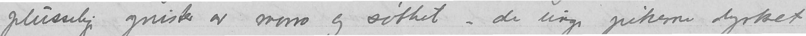plutselige gnister av morro og søthet – de unge pikerne dyrket
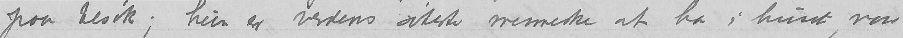paa besøk; hun er verdens søteste menneske at ha i huset, naar
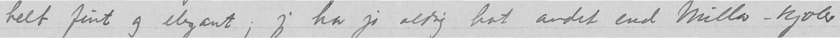helt fint og elegant;, jeg har jo aldrig hat andet endn Müller…-kjoler
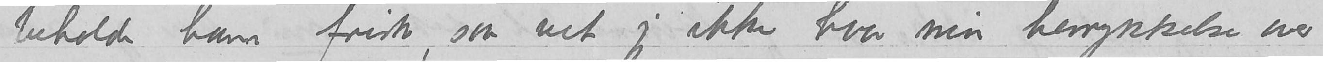beholde ham frisk, saa vet jeg ikke hvor min henrykkelse over
